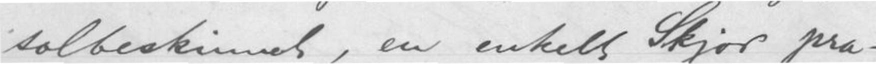solbeskinnet, en enkelt Skjor pra-
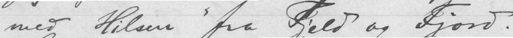med Hilsen "fra Fjeld og Fjord."
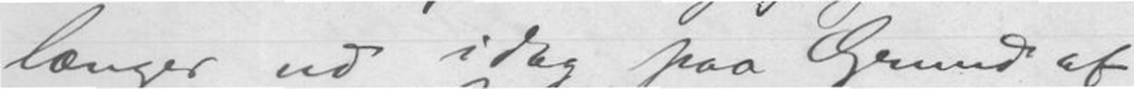længer ud idag paa Grund af
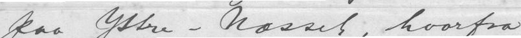paa Ytre-Næsset, hvorfra
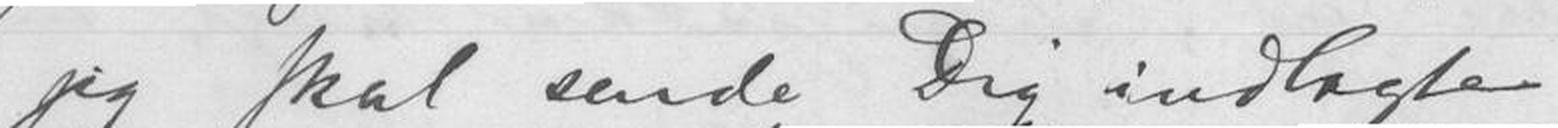jeg skal sende Dig indlagte
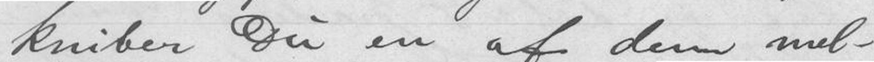kniber Du en af dem mel-
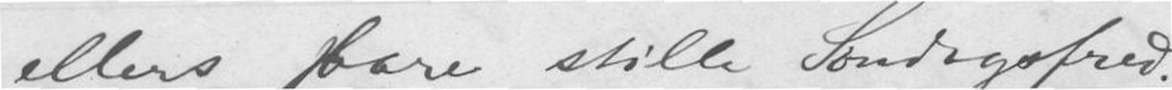ellers bare stille Søndagsfred.
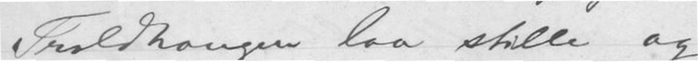Troldhaugen laa stille og
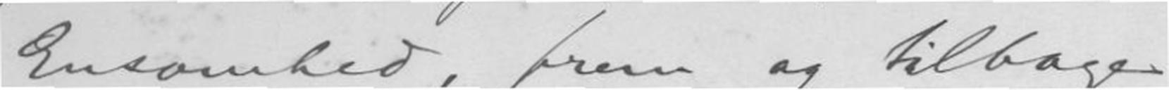Ensomhed, frem og tilbage
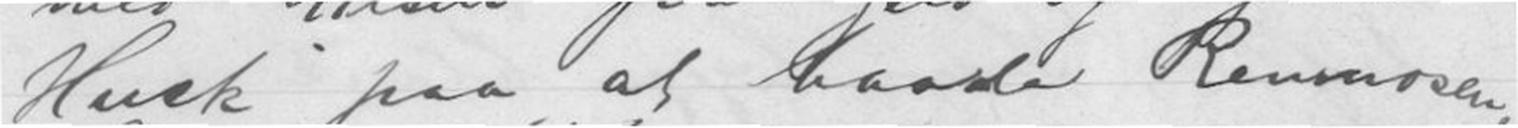Husk paa at baade Renmosen,
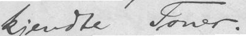kjendte Toner. -
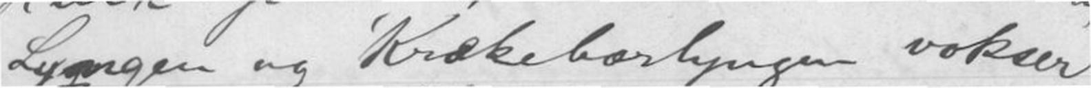Lyngen og Krækebærlyngen vokser
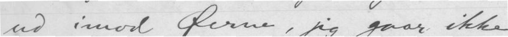ud imod Øerne, jeg gaar ikke
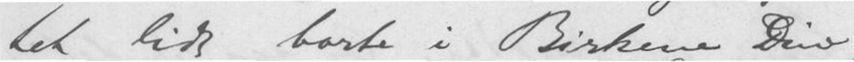tet lidt borte i Birkene Dine,
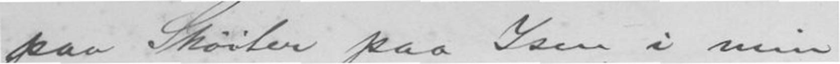paa Skøiter paa Isen i min
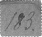183.
